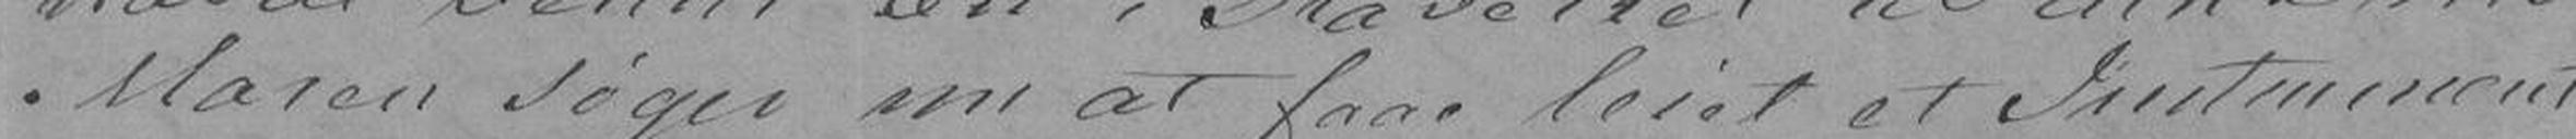Maren søger nu at faae leiet et Instrument
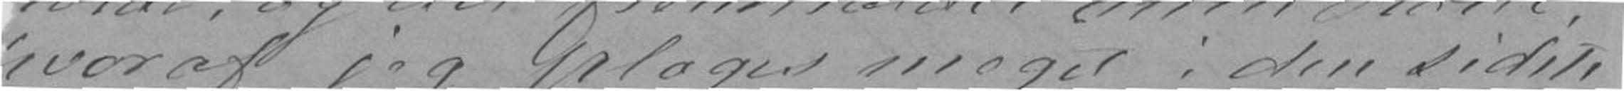hvoraf jeg plages meget i den sidste
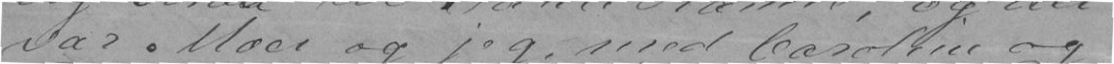var Moer og jeg med Caroline og
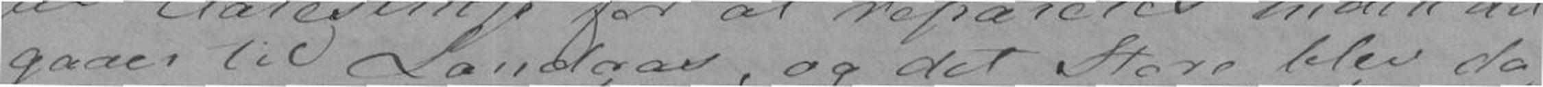gaar til Landaas, og det Store blev da
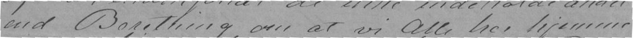end Beretning om at vi Alle her hjemme
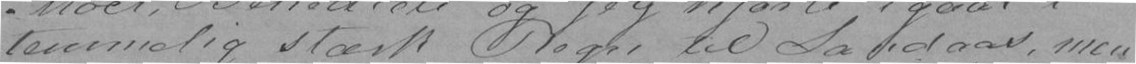temmelig stærk Regn til Landaas, men
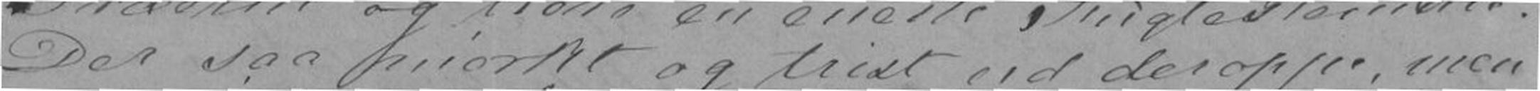Der saa mørket og trist ud deroppe, men
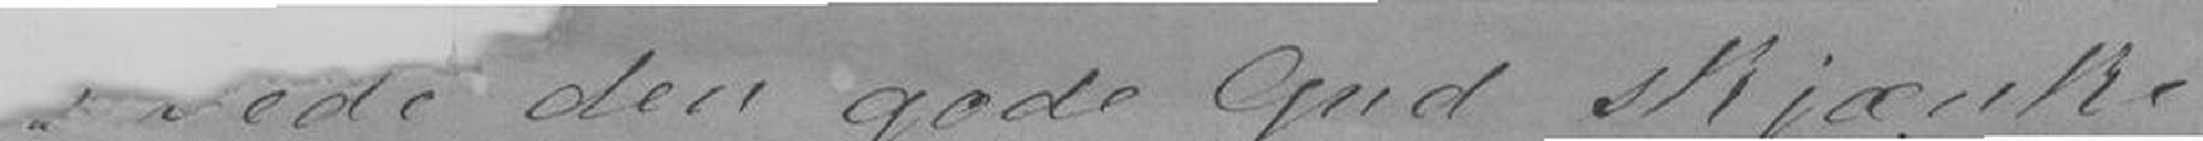vede den gode Gud skjænke
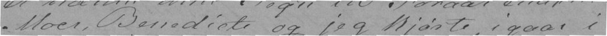Moer, Benedicte og jeg kjørte igaar i
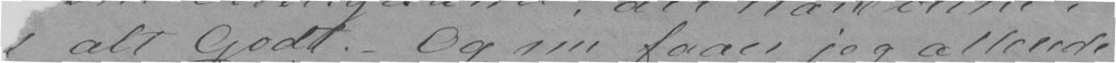alt Godt. - Og nu faaer jeg allerede
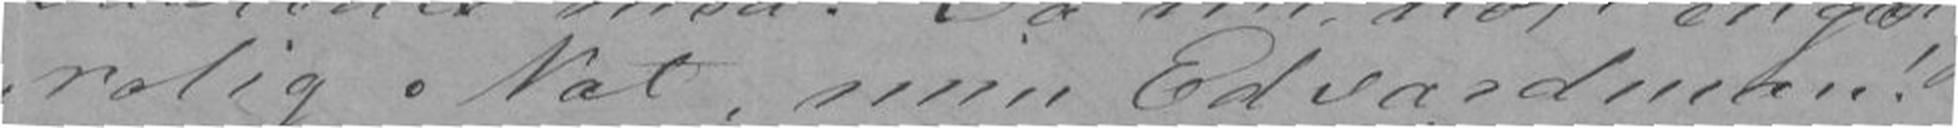rolig Nat, min Edvardman!
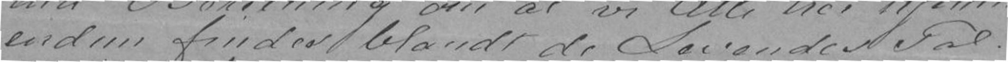endnu findes blandt de Levendes Tal.
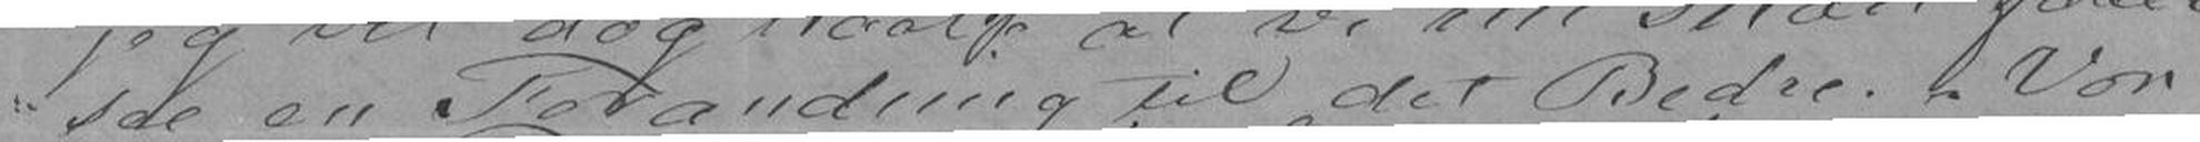see en Forandring til det Bedre. Vor
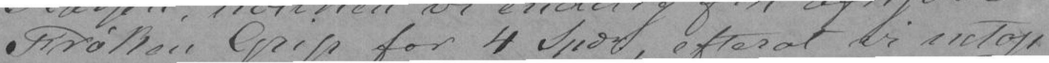Frøken Grip for 4 Spd., efterat vi netop
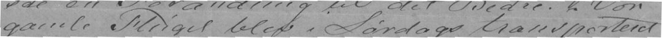gamle Flügel blev i Lørdags transporteret
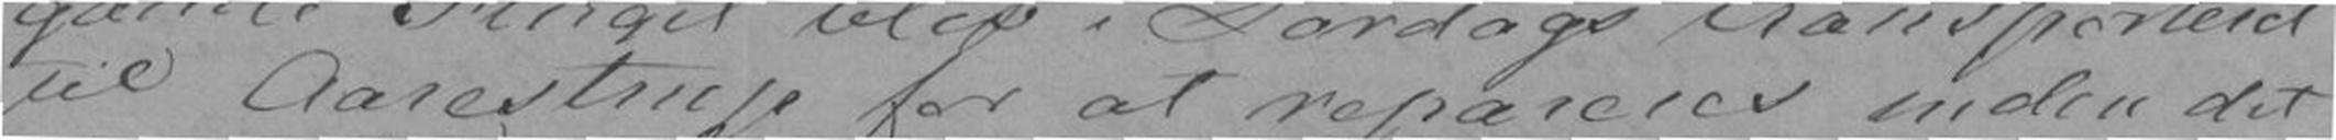til Aarestrup for at repareres inden det
